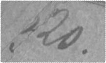120.
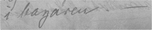i bagaren. -
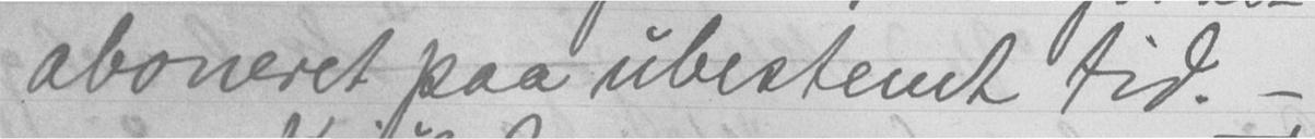aboneret paa ubestemt tid. -
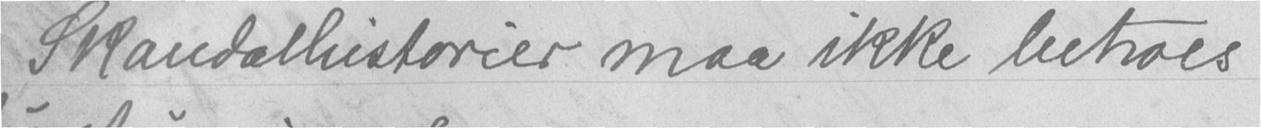- Skandalhistorier maa ikke betroes
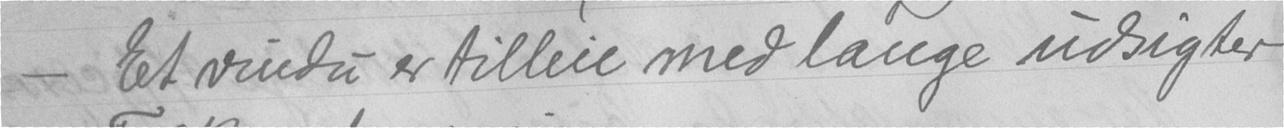- Et vindu er tilleie med lange udsigter
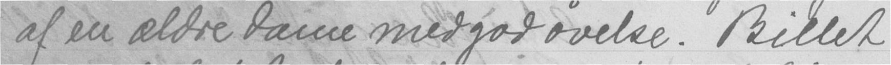af en ældre dame med god øvelse. Billet
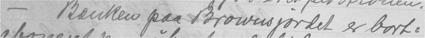- Bænken paa Brownsjordet er bort-
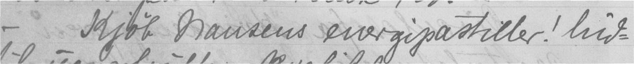- Kjøb Nansens energipastiller! hid-
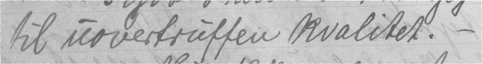til uovertruffen kvalitet. -
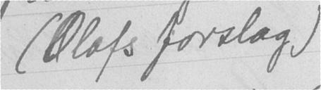(Olafs forslag)
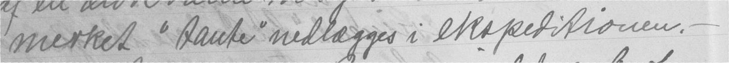merket "tante" nedlægges i ekspeditionen. -
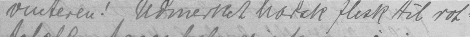vinteren! Udmerket harsk flesk til rot-
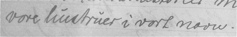vore hustruer i vort navn.
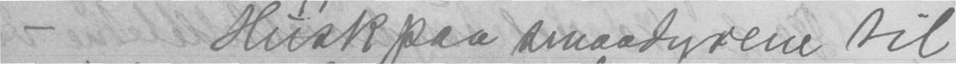- Husk paa smaadyrene til
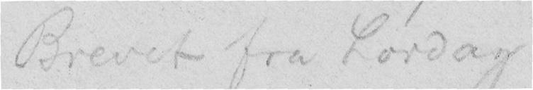Brevet fra Lørdag
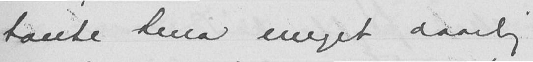tante Lena meget daarlig
